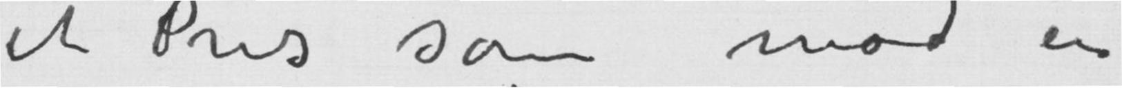et Pus som mod en
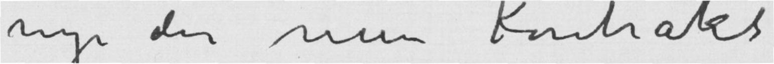nye der men Kontrakt
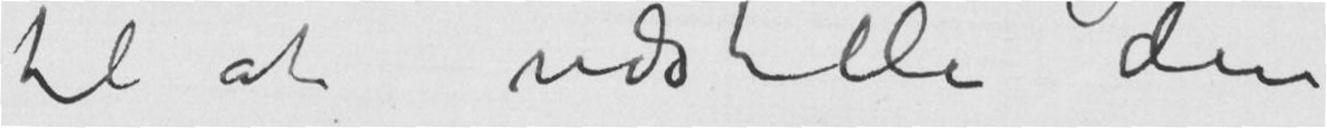til at udstille den
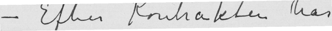– Efter Kontrakten har
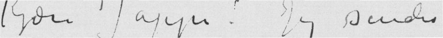Kjære Jappe! Jeg sender
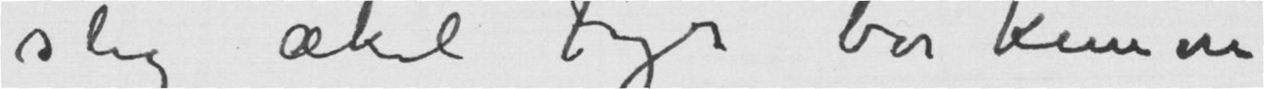slig ækel Fyr bør kunne
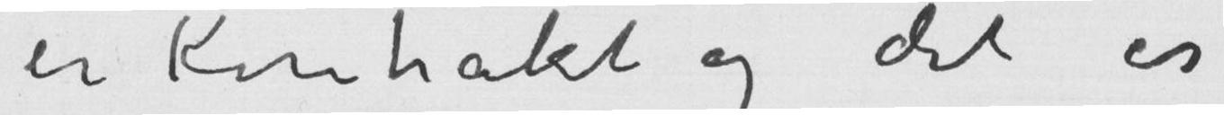er Kontrakt og det er
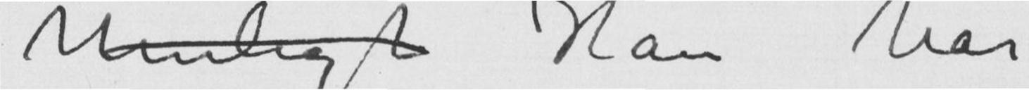Muligt Han har
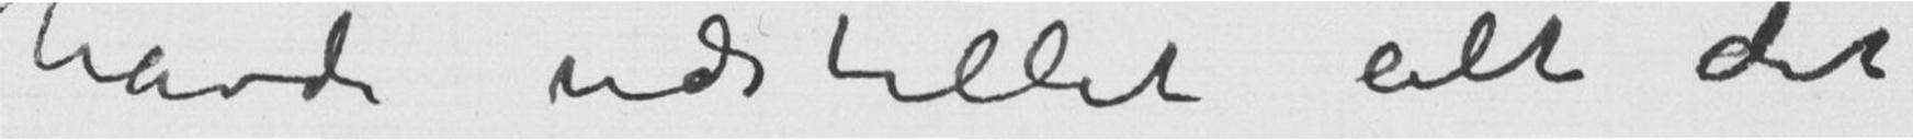havde udstillet alt det
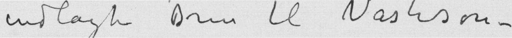indlagte brev til Vasteson –
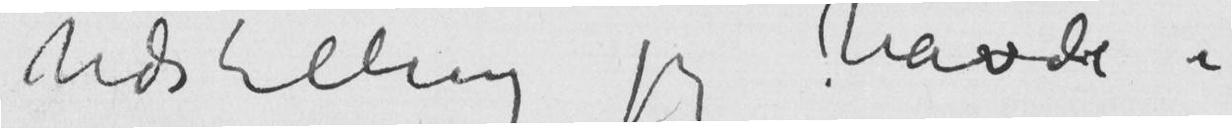Udstilling jeg havde i
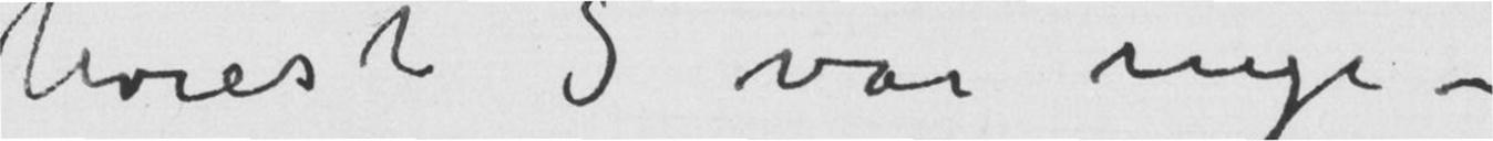høiest 5 var nye –
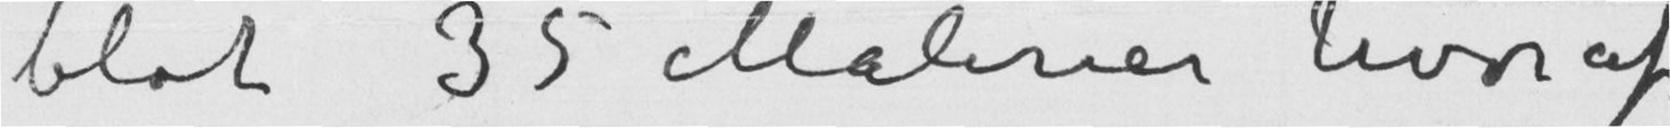blot 35 Malerier hvoraf
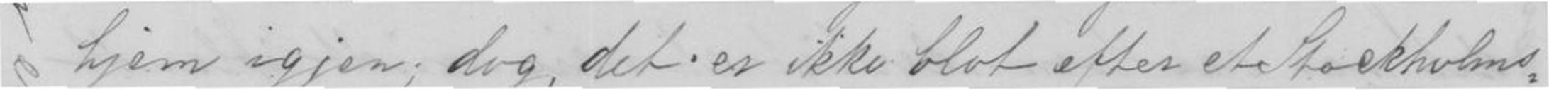hjem igjen; dog det er ikke blot efter et Stockholms
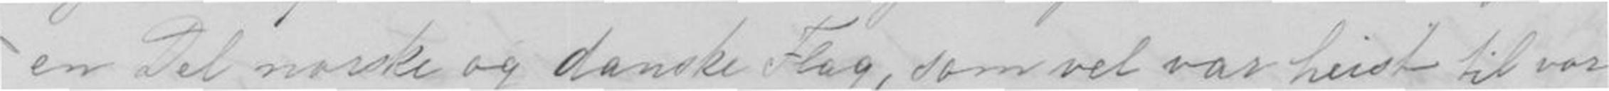en Tel maske og danske flag, som vel var hvist til vor
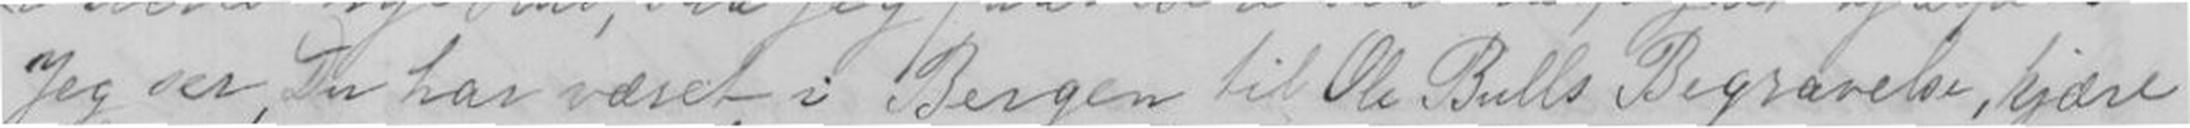Jeg ser, Du har været i Bergen til Ole Bulls Begravelse, kjære
